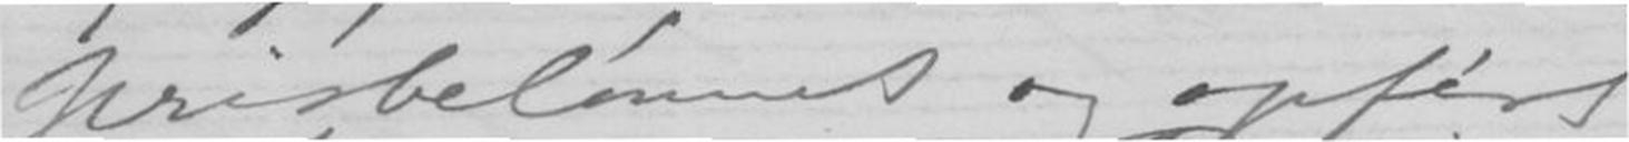prisbelønnet og opført
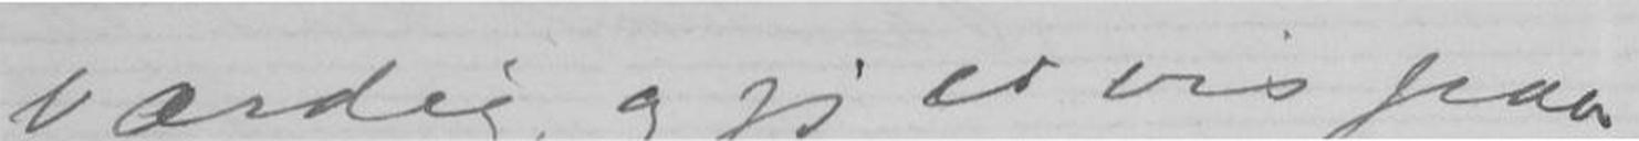værdig og jeg er vis paa
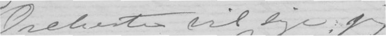Orchester vil sige jeg
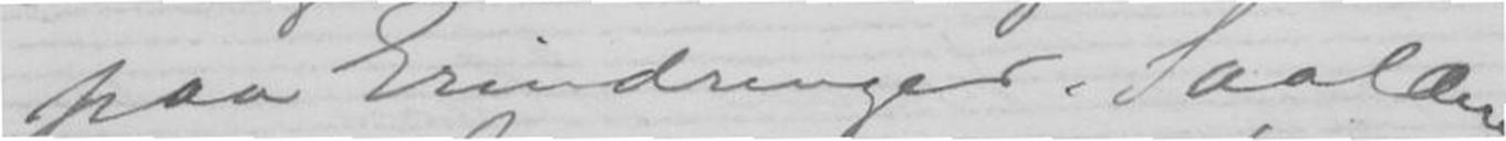Erindringer. Saalænge
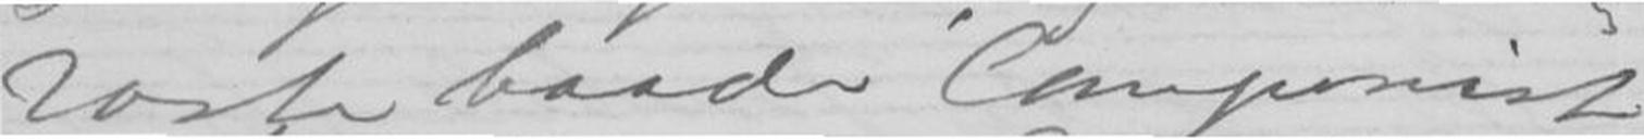roste baade Componist
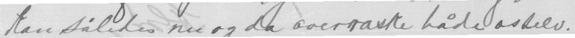kan således nu og da overraske både os selv
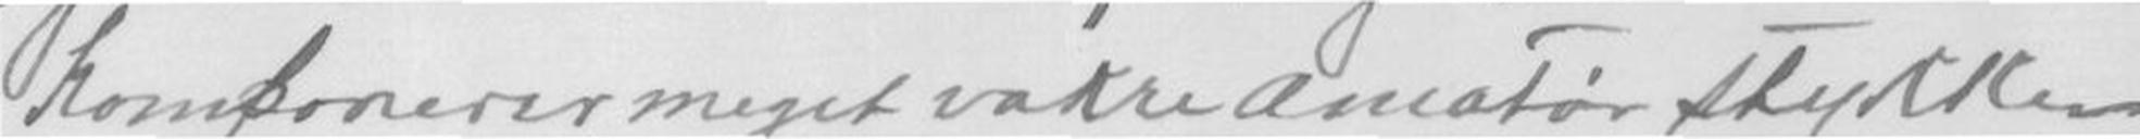komponerer meget vakre Amatør stykker.
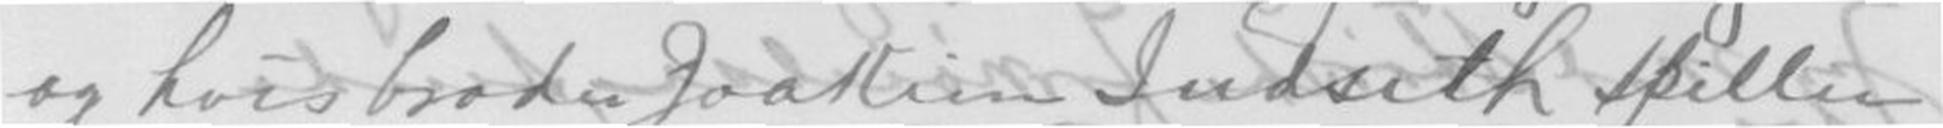og hvis broder Joakim Indseth spiller
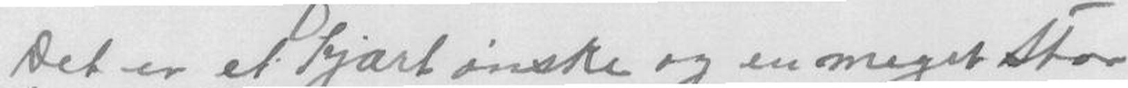Det er et kjært ønske og en meget stor
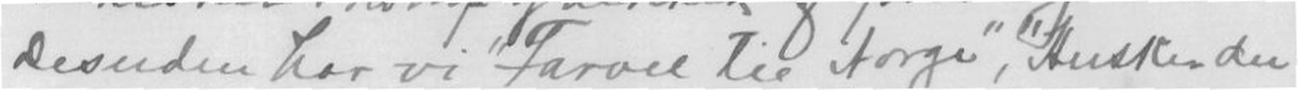Desuden har vi "Farvel til Norge", "Husker du
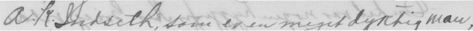A.K.Indseth, som er en meget dygtig man,
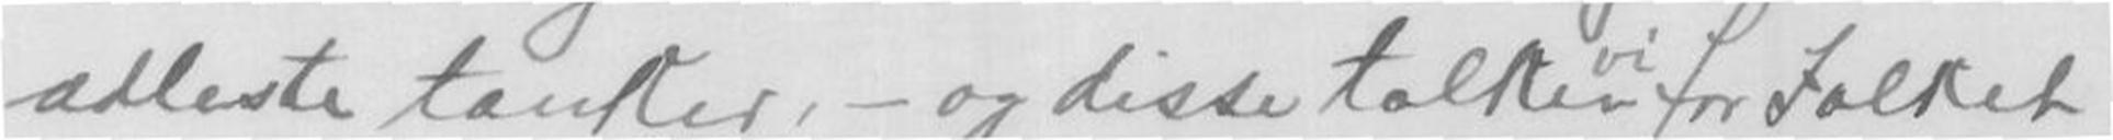adleste tanker, - og disse tolker vi for Folket
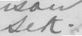Sek.
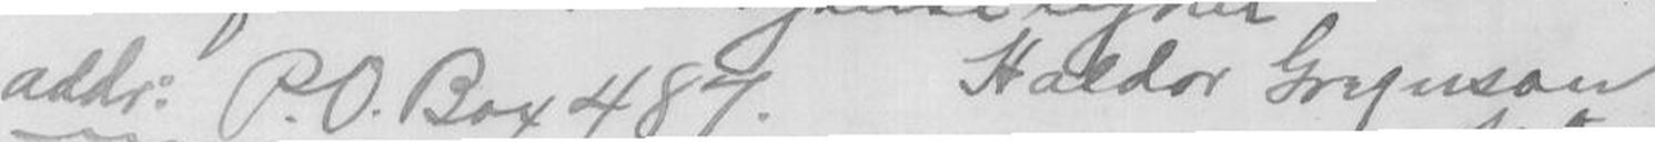addr: P.O.Box 487. Haldor Greguson
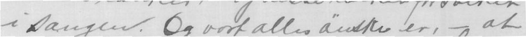i Sangen. Og vort alles ønske er, - at
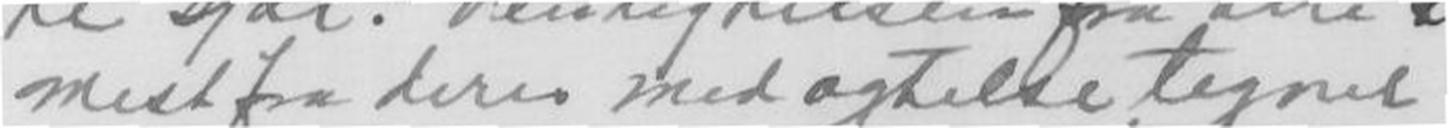mest fra deres med agtelse tegner
